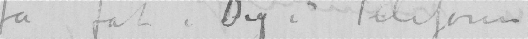få fat i Dig i Telefonen
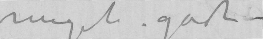meget godt.
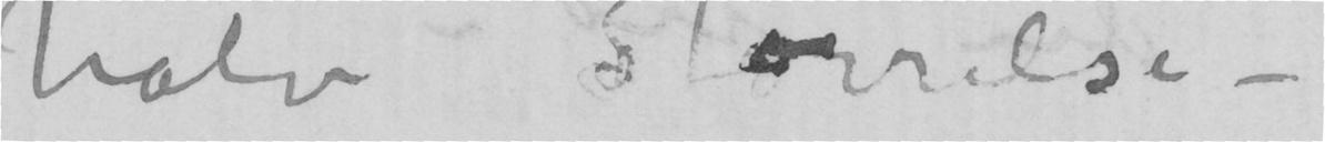halv Størrelse –
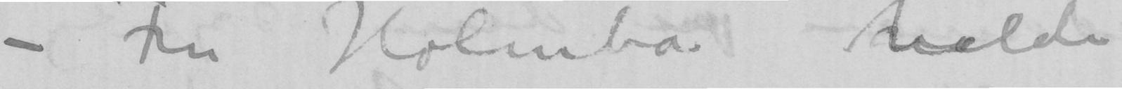– Fru Holmboe vilde
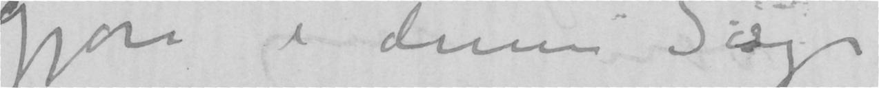gjøre i denne Sag
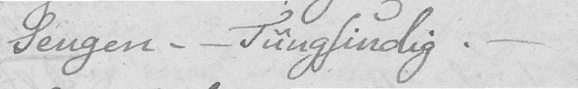Sengen. – Tungsindig. –
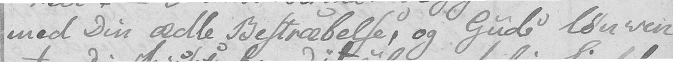med Din ædle Bestræbelse, og Guds løn ven
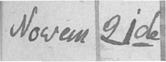Novem 21de
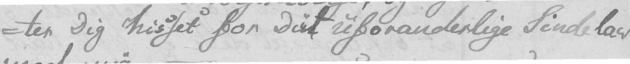-ter Dig hisset for Dit uforanderlige Sindelav
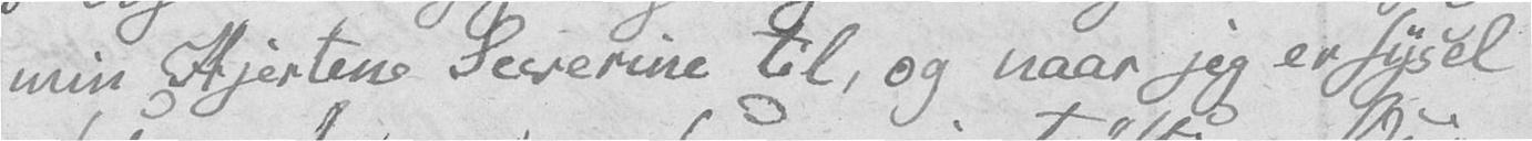min Hjertens Severine til, og naar jeg er sysel
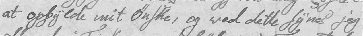at opfylde mit Ønske, og ved dette synes jeg
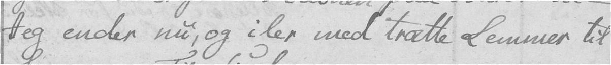Jeg ender nu, og iler med trætte Lemmer til
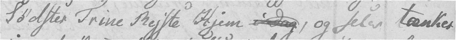Sødster Trine Reiste Hjem i Dag, og selv tænker
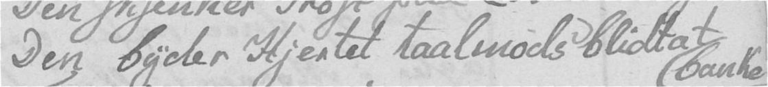Den byder Hjertet taalmodsblidt at banke
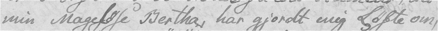min Mageløse Bertha, har gjordt mig Løfte om,
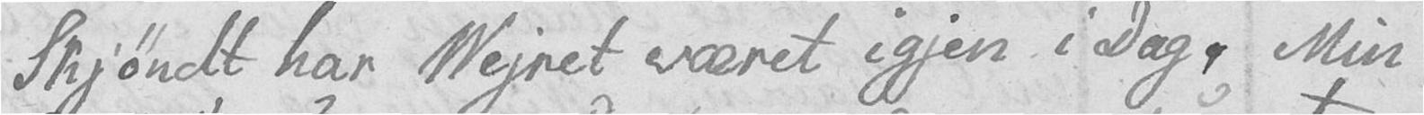Skjøndt har Vejret været igjen i Dag. Min
